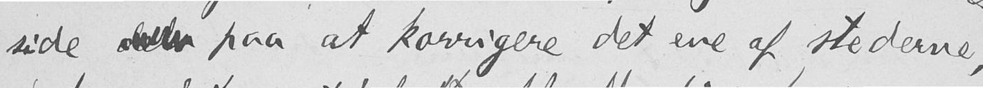side  paa at korrigere det ene af stederne,
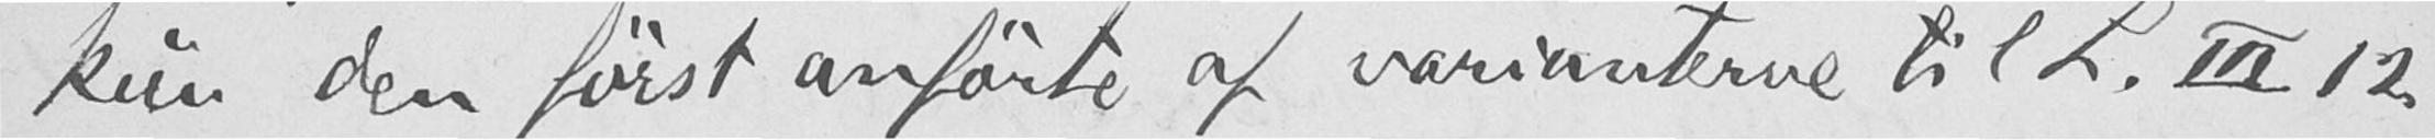kun den først anførte af varianterne til L. III 12
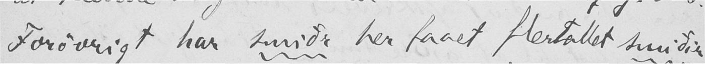Forøvrigt har smiðr her faaet flertallet smiðir,
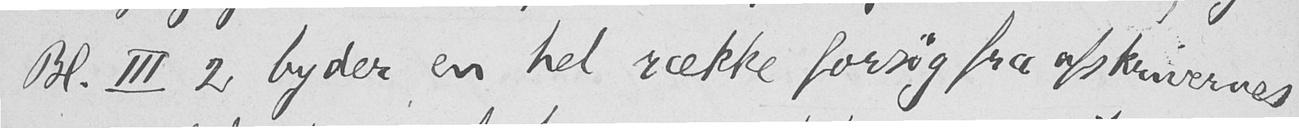Bl. III 2 byder en hel række forsøg fra afskrivernes
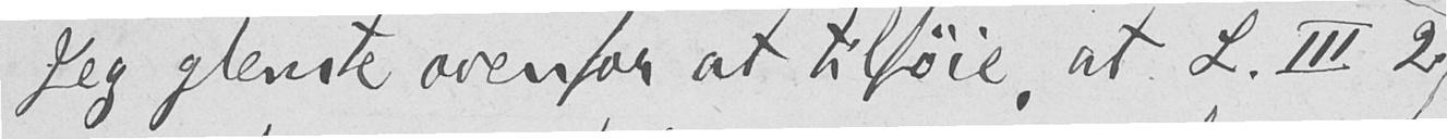Jeg glemte ovenfor at tilføie, at L. III 2
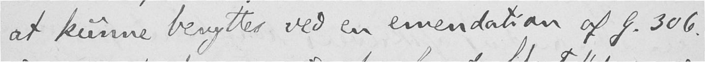at kunne benyttes ved en emendation af G. 306.
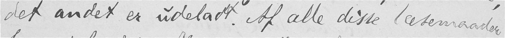det andet er udeladt. Af alle disse læsemaader,
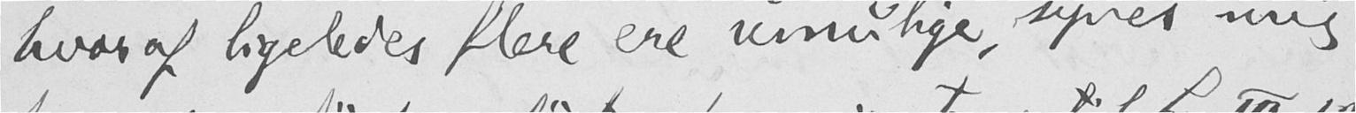hvoraf ligeledes flere ere umulige, synes mig
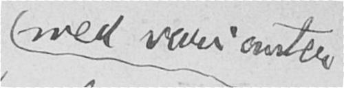med varianter
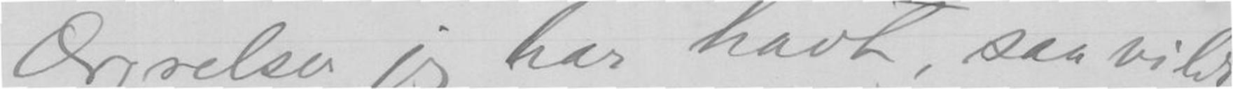Ærgrelser jeg har havt, saa vilet
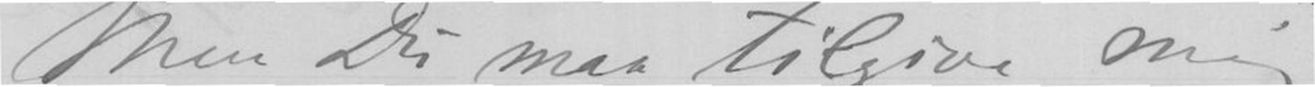Men Du maa tilgive mig
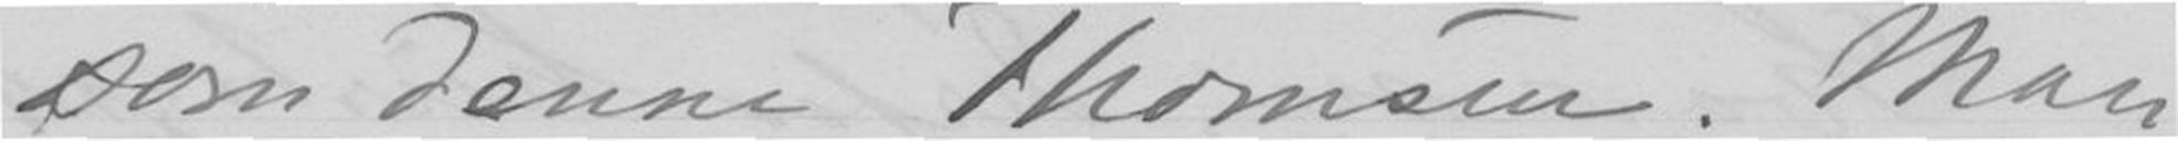som denne Thomsen. Man
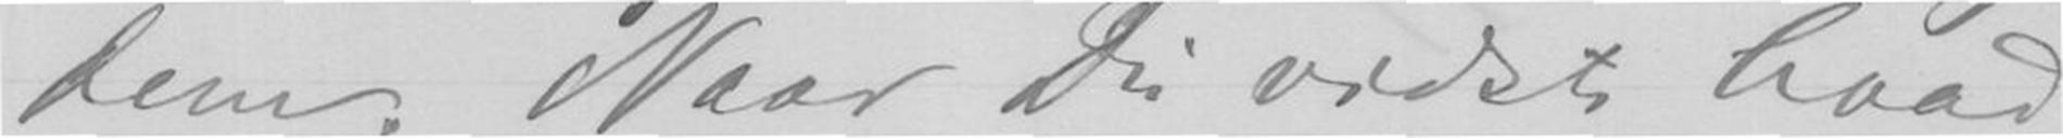dem. Naar Du vidste hvad
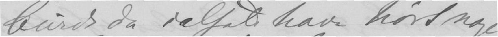burde da ialtfald have hørt noget
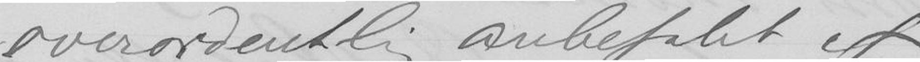overordentlig anbefalet af
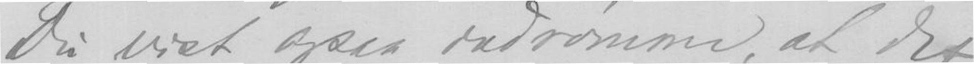Du vist ogsaa indrømme, at det
